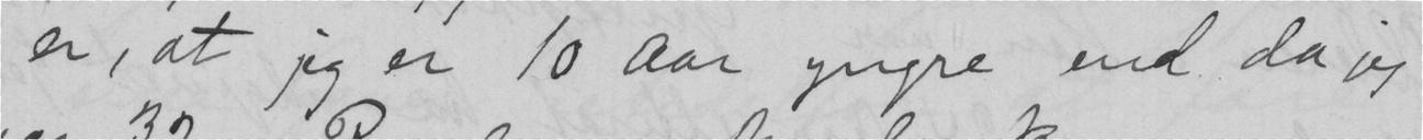er, at jeg er 10 Aar yngre end da jeg
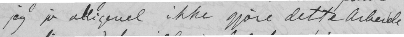jeg jo alligevel ikke gjøre detta arbeide
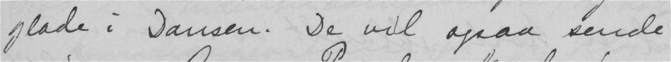glade i Dansen. De vil ogsaa sende
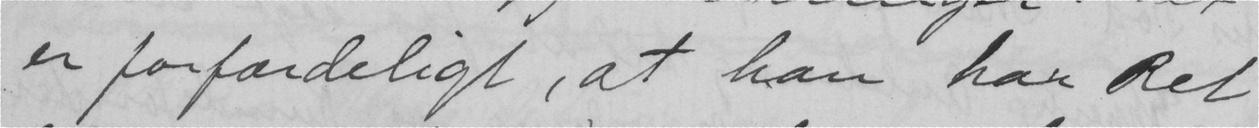er forfærdeligt, at han har Ret
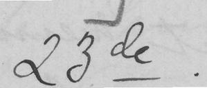23de.
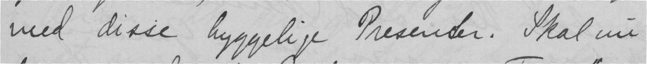med disse hyggelige Presenter. Skal nu
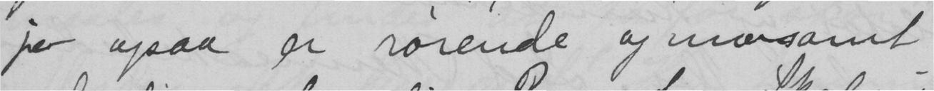jo ogsaa er rørende og morsomt
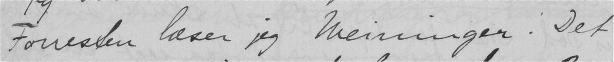Forresten læser jeg Weininger. Det
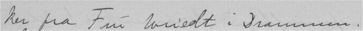ker fra Fru Wriedt i Drammen.
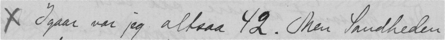x Igaar var jeg altsaa 42. Men Sandheden
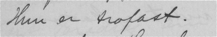Hun er trofast.
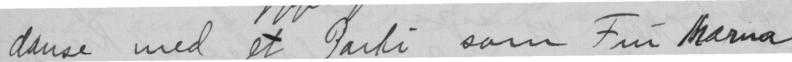danse med et Parti som Fru Marna
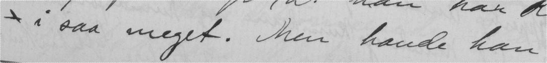i saa meget. Men havde han
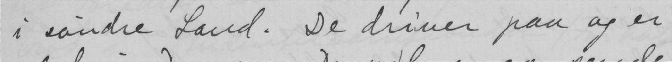i søndre Land. De driver paa og er
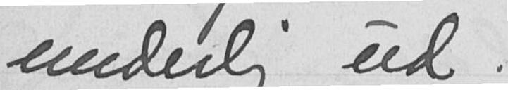underlig ud.
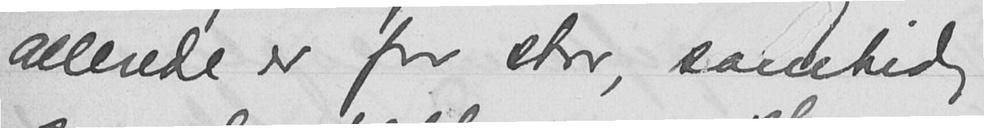allerede er for stor, samtidig
